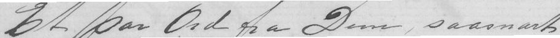Et par Ord fra Dem, saasnart
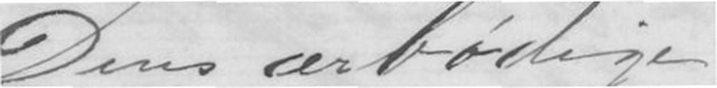Deres ærbødige
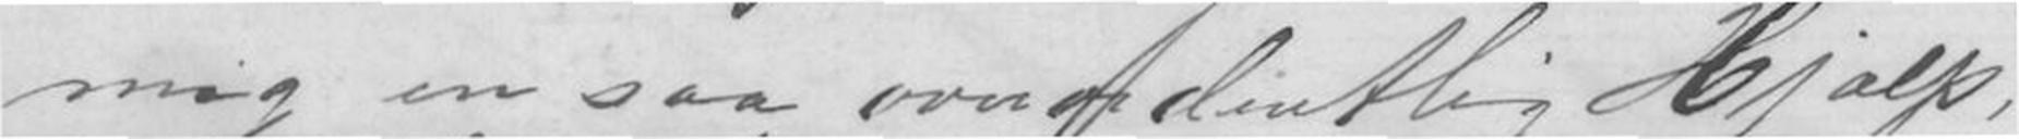mig en saa overordentlig Hjælp,
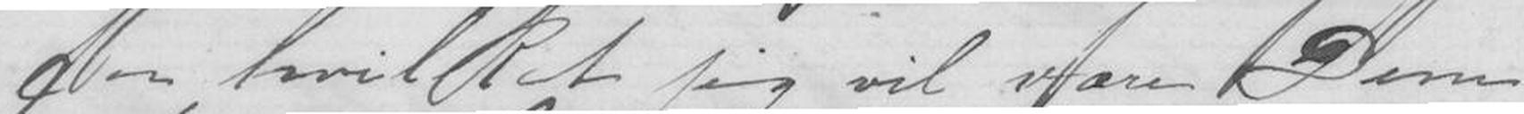som hvilket jeg vil være Dem
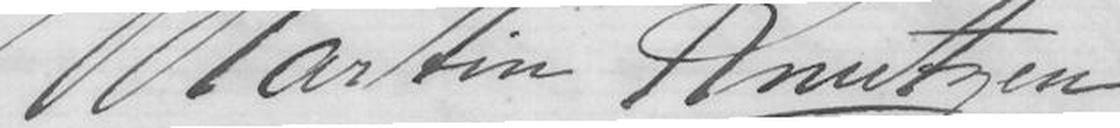Martin Knutzen
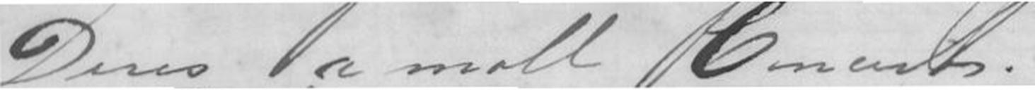Deres a moll Concert.
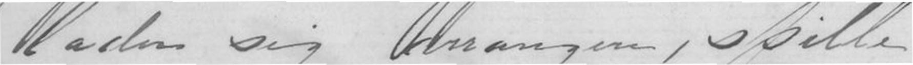lader sig arrangere, spille
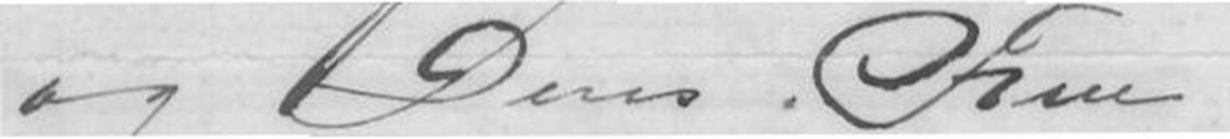og Deres Frue
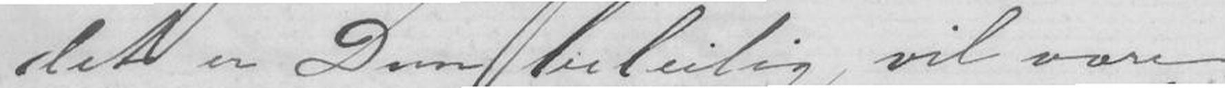det er Dem beleilig, vil være
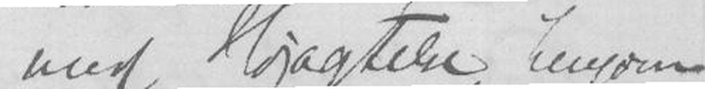med Højagtelse hengivne
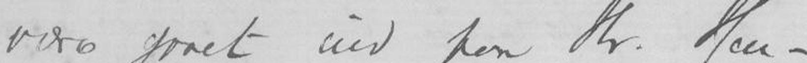være gaaet ud paa Hr. Heu-
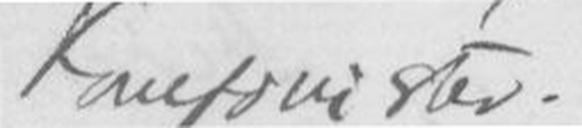Komponisten.
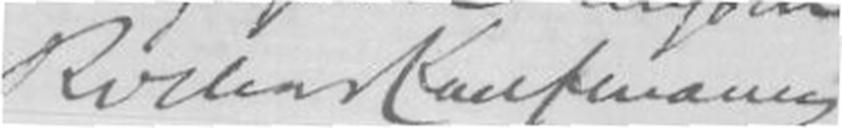Richard Kaufmann
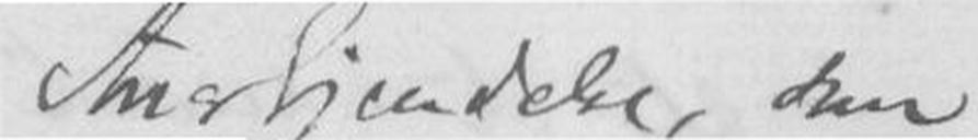den Anskjendelse, som
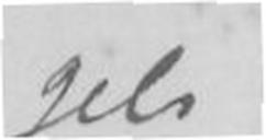gels
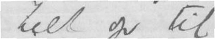helt op til
